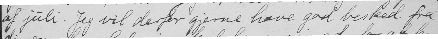af juli. Jeg vil derfor gjerne have god besked fra
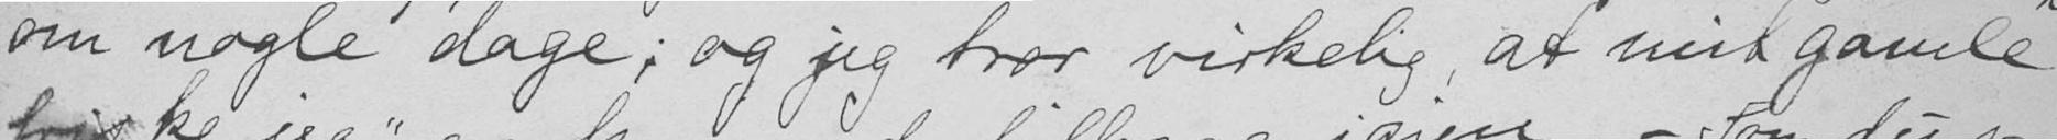om nogle dage; og jeg tror virkelig, at mit gamle
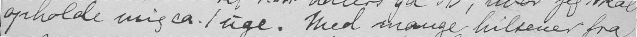opholde mig ca. 1 uge. Med mange hilsener fra
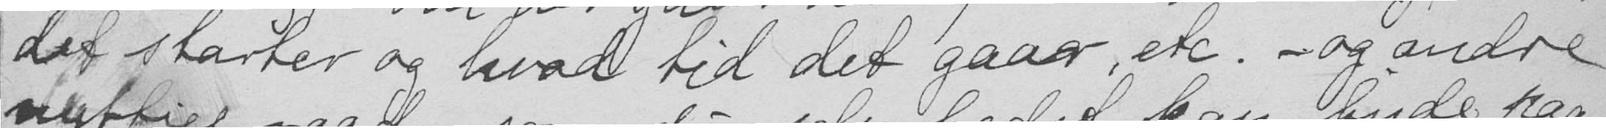det starter og hvad tid det gaar, etc. - og andre
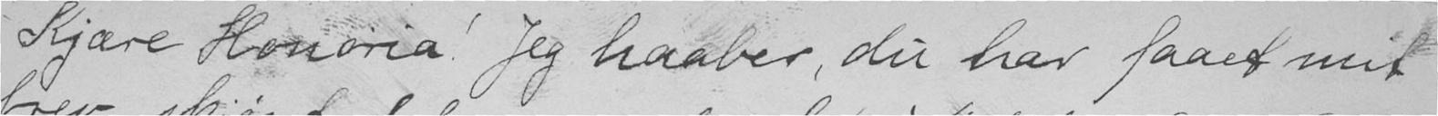Kjære Honoria. Jeg haaber, du har faaet mit
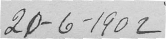20-6-1902
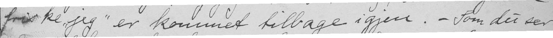friske jeg er kommet tilbage igjen. - Som du ser
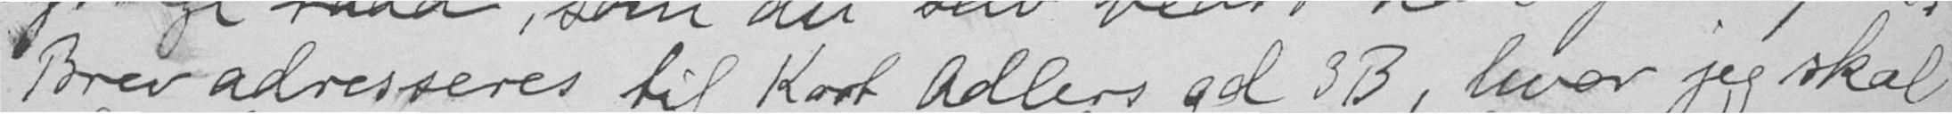Brev adresseres til Kort Adlers gd 3B, hvor jeg skal
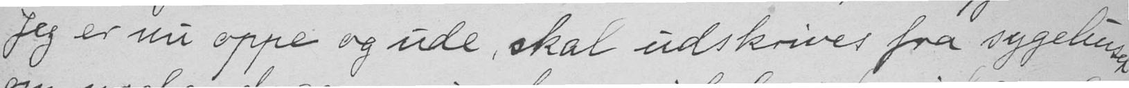Jeg er nu oppe og ude, skal udskrives fra sygehuset
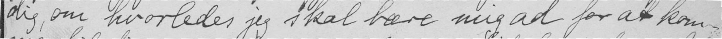dig, om hvorledes jeg skal bære mig ad for at kom-
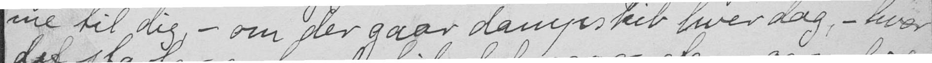me til dig, - om der gaar dampskib hver dag, - hvor
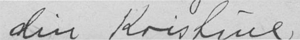din Kristine.
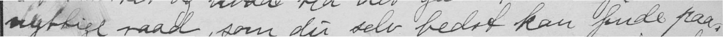nyttige raad, som du selv bedst kan finde paa.
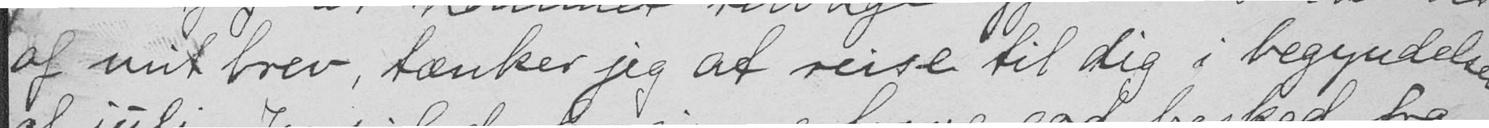af mit brev tænker jeg at reise til dig i begyndelsen
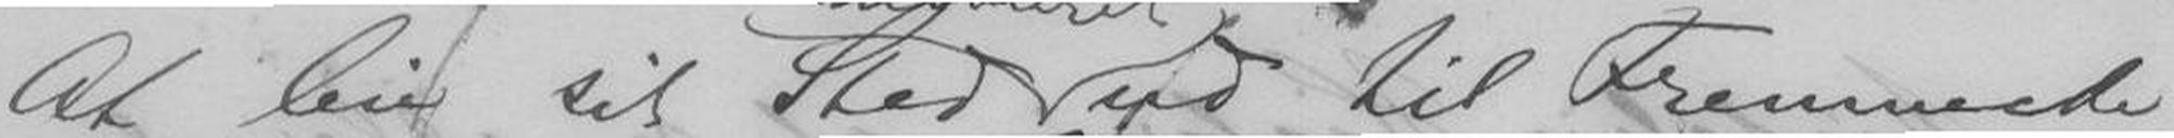At leie sit Sted ud til Fremmede
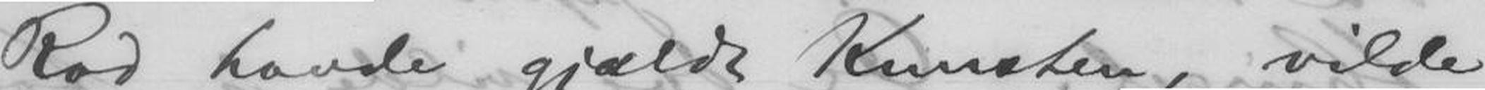Rod havde gjældt Kunsten, vilde
